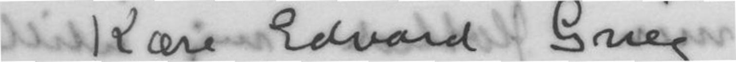Kære Edvard Grieg!
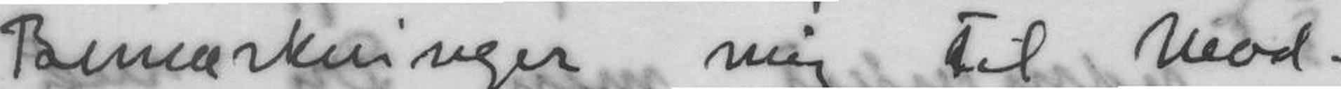Bemærkninger mig til Mod-
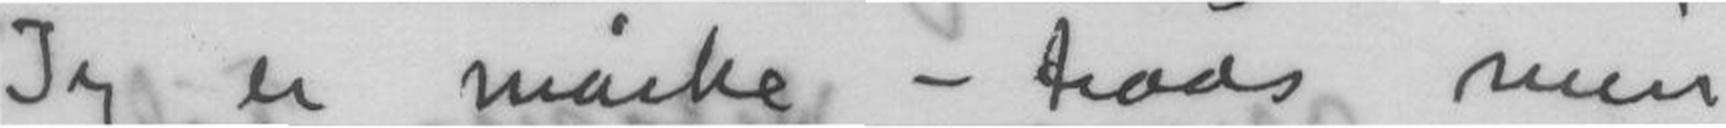Jeg er måske - trods min
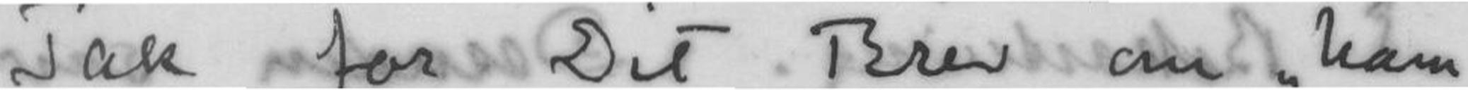Tak for Dit Brev om "ham
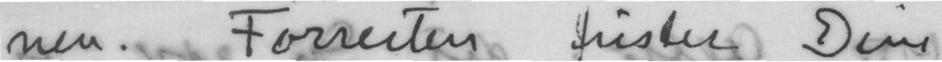nen. Foresten fristes Dine
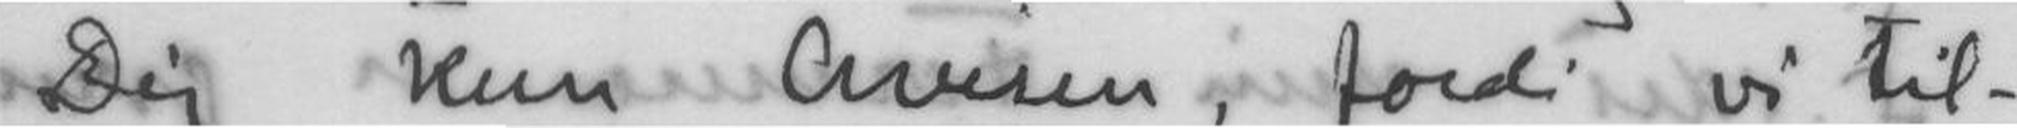Dig kun Avisen, fordi vi til-
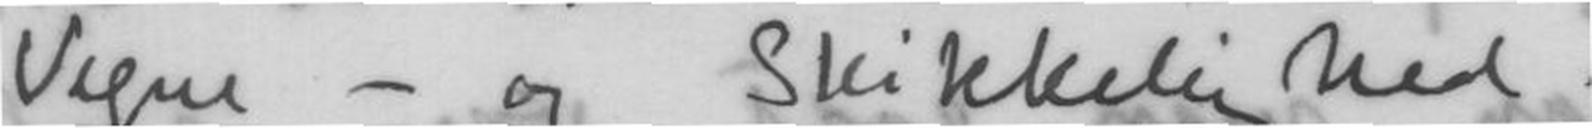Vegne - og Skikkelighed.
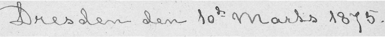Dresden den 10de Marts 1875.
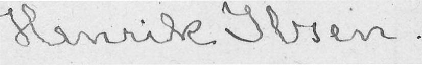Henrik Ibsen.
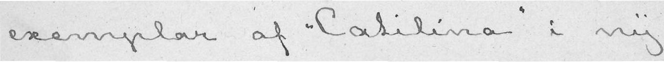exemplar af «Catilina» i ny
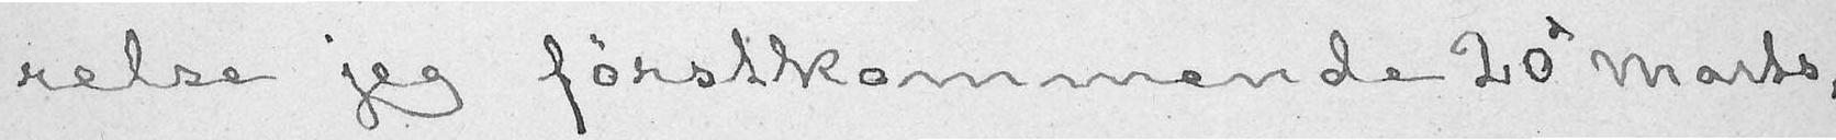relse jeg førstkommende 20de Marts,
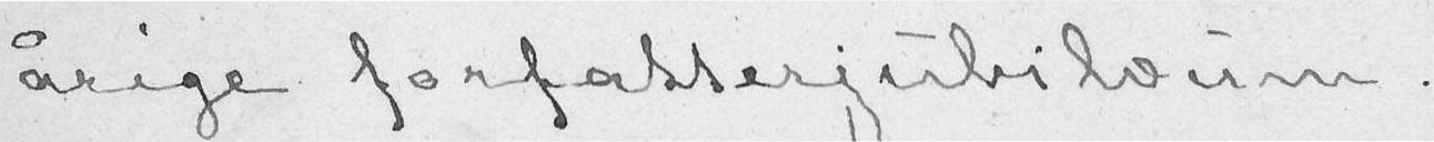årige forfatterjubileæum.
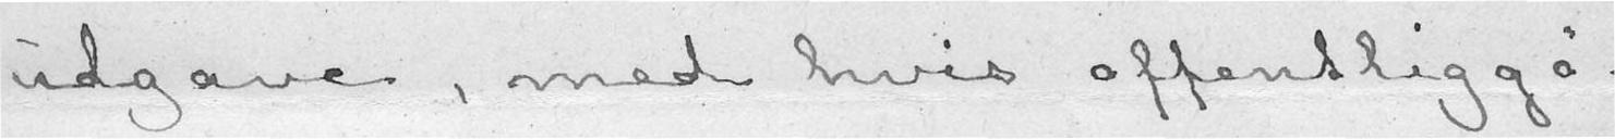udgave, med hvis offentliggø-
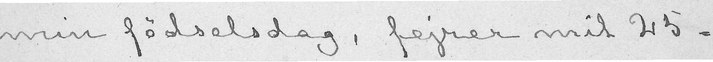min fødselsdag, fejrer mit 25-
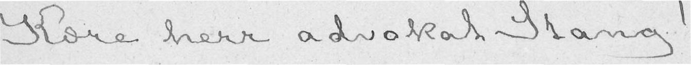Kære herr advokat Stang!
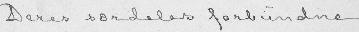Deres særdeles forbundne
